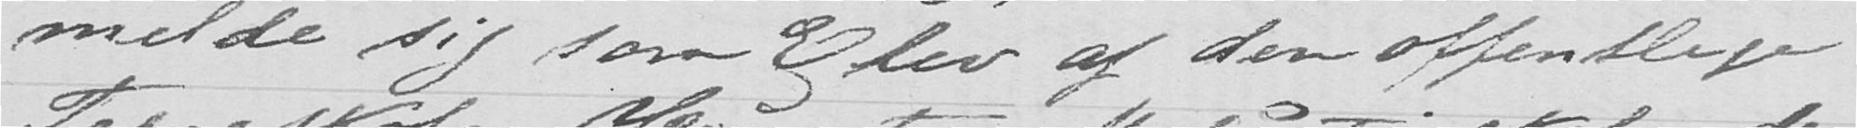melde sig som Elev af den offentlige
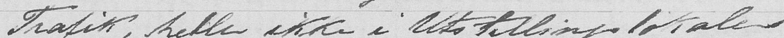Trafik, heller ikke i Utstillingslokalers
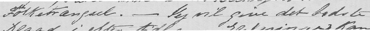Folketrængsel. - Jeg vil give det bedste
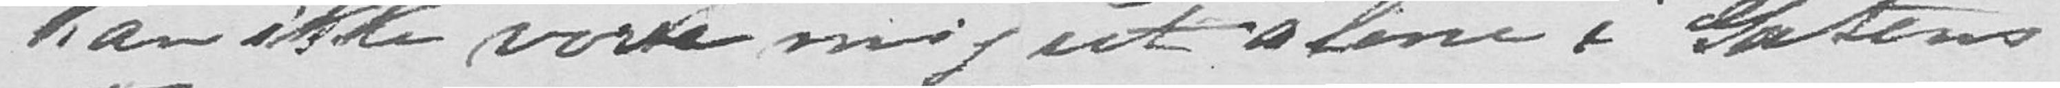kan ikke vorte mig utt alene i Gatens
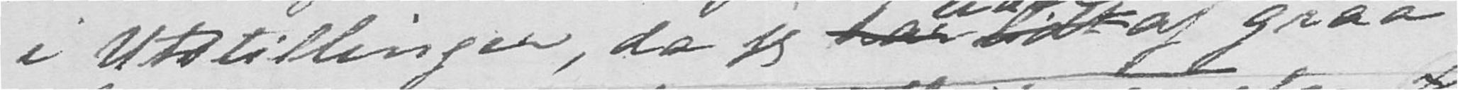i Utstillinger, da jeg har lidt af graa
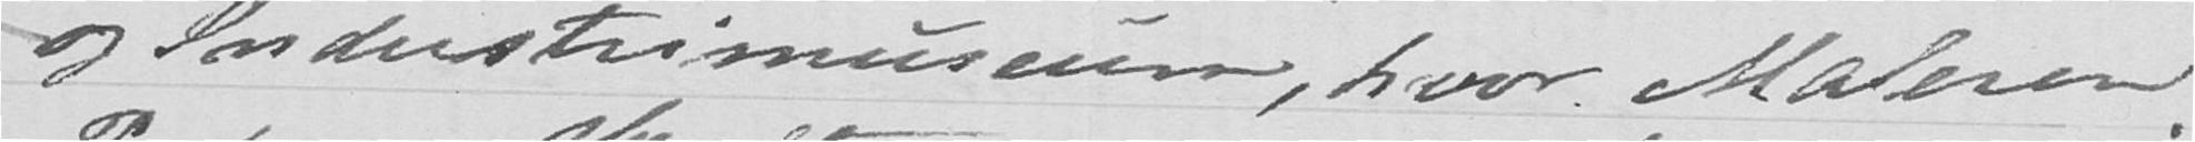og Industrimuseum, hvor Maleren
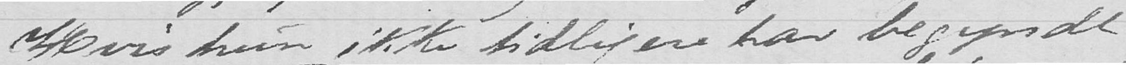Hvis hun ikke tidligere har begyndt
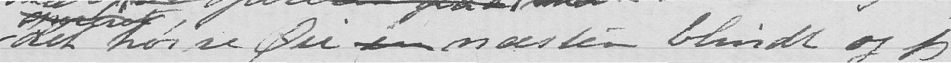det høire Øie en næsten blindt og jeg
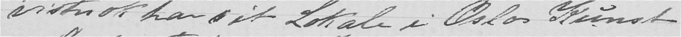vistnok har sit Lokale i Oslos Kunst
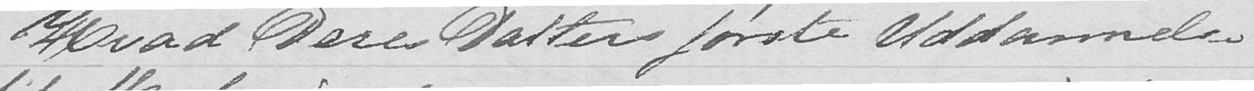Hvad Deres Datters første Uddannelse
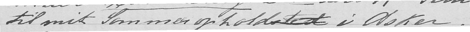til mit Sommeropholdsted i Asker.
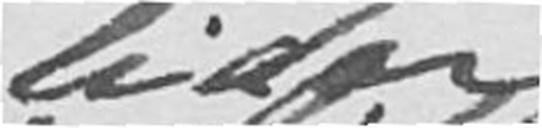lider
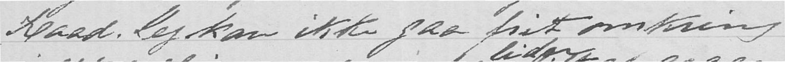Raad. Jeg kan ikke gaa frit omkring
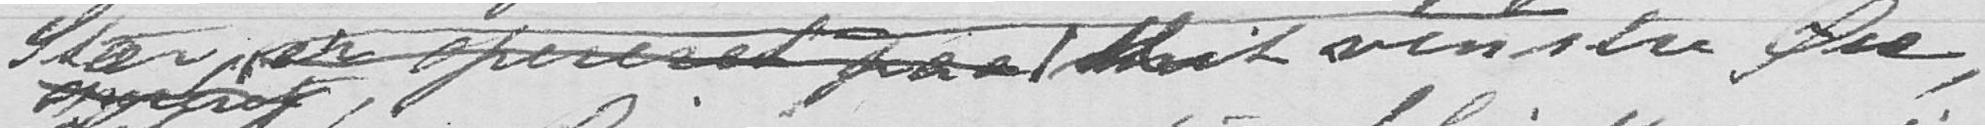Stær, er opereret paa det venstre Øie
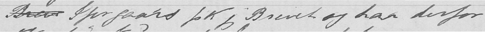Brev I forgaars fik jeg Brevet og har derfor
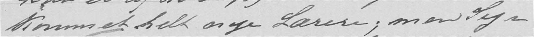kommet helt nye Lærere; men Sy-
